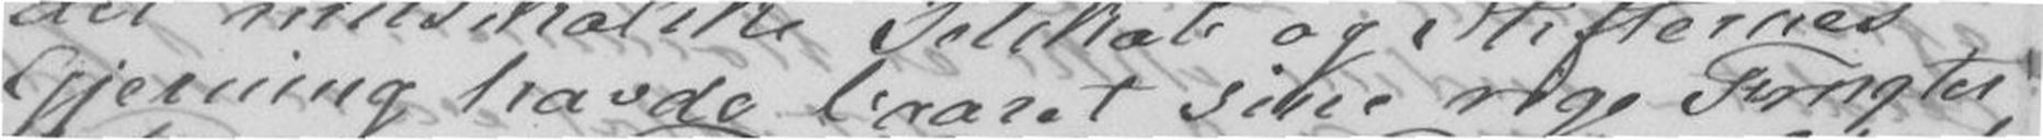gjerning havde baaret sine rige Frugter
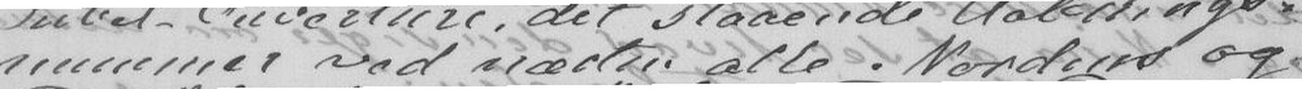nummer ved næsten alle Nordens og
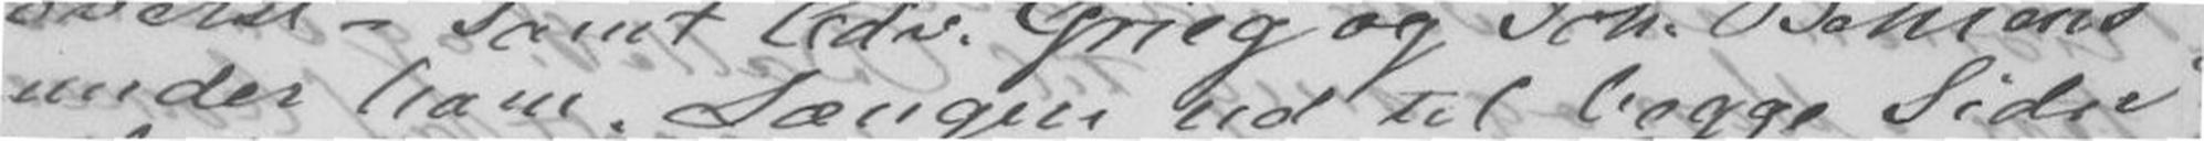under ham. Længere ud til begge Sider
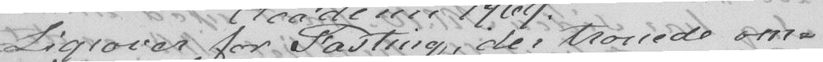Ligeover for Fasting, der troede om-
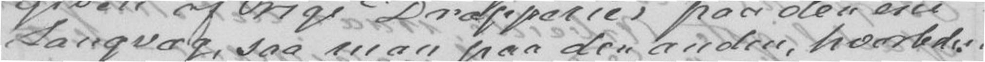Landvæg, saa man paa den anden hvorledes
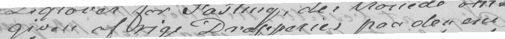given af rige Dræpperies paa den ene
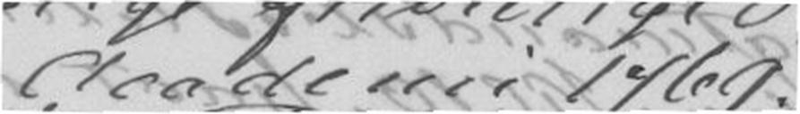Academi 1769.
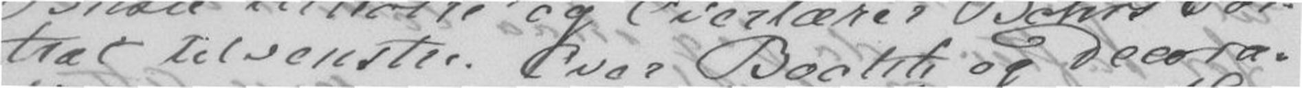træt tilvenstre. Over Boalth og Decora-
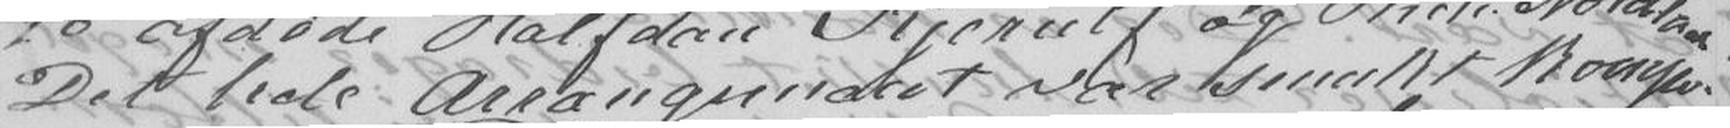Det hele Arrangementet var smukt kompo-
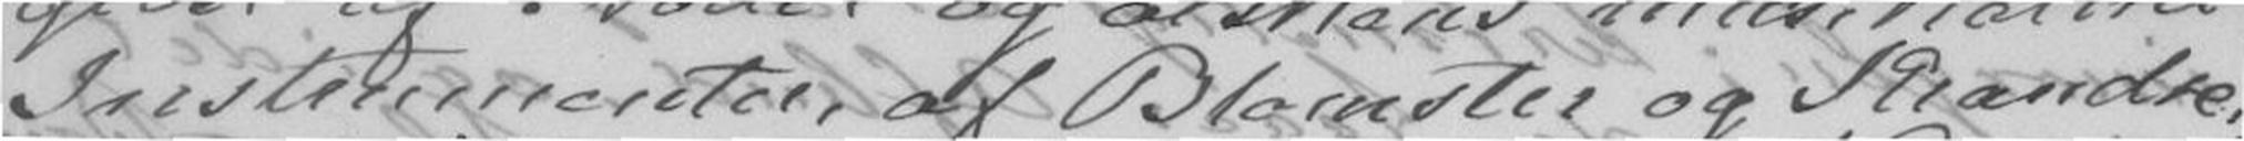Instrumenter, af Blomster og Krandse,
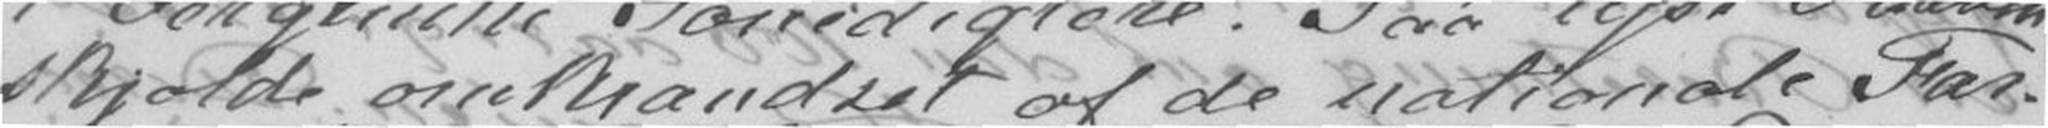skjolde omkrandset af de nationale Far-
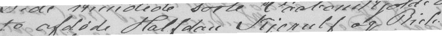to afdøde Halfdan Kjerulf og Rich.
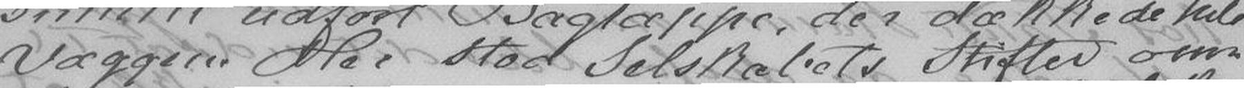Væggen. Her stod Selskabets Stifter om-
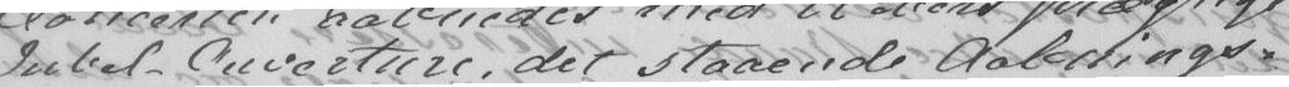Jubel-Overture, det staaende Aabnings-
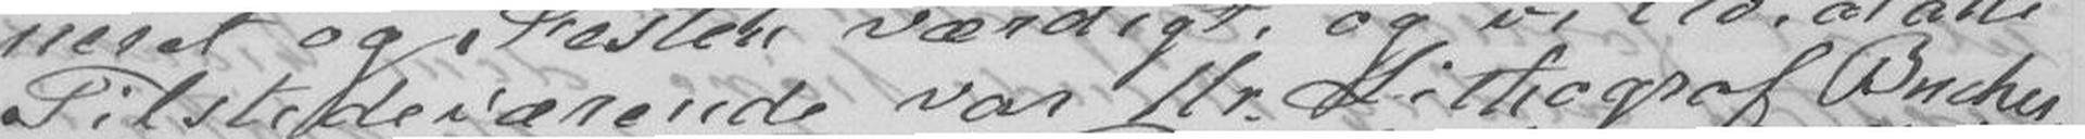Tilstedeværende var Hr. Lithograf Buches,
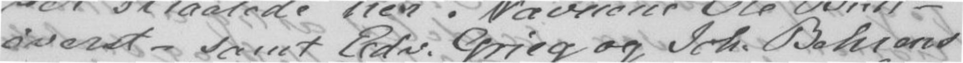øverst - samt Edv. Grieg og Joh. Behrens
